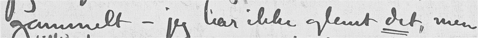gammelt - jeg har ikke glemt det, men
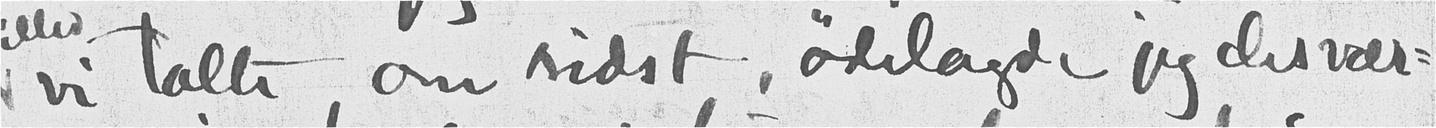vi talte om sidst, ødelagde jeg desvær-
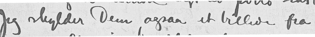Jeg skylder Dem ogsaa et billede fra
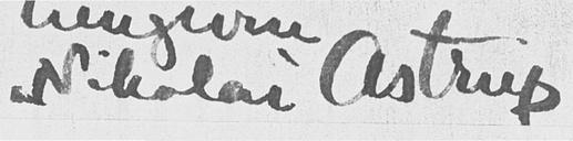Nikolai Astrup
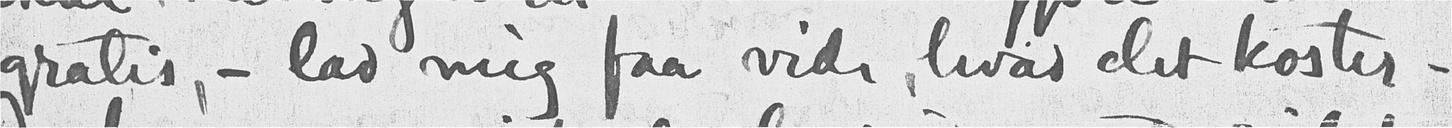gratis, - lad mig faa vide, hvad det koster -
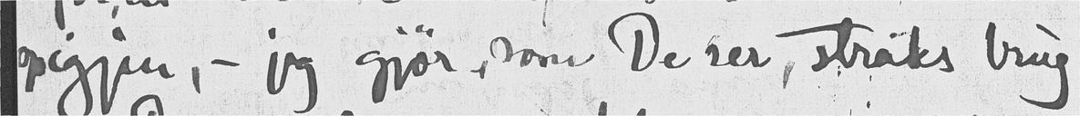op igjen, - jeg gjør, som De ser, straks brug
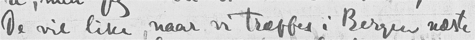De vil like, naar vi træffes i Bergen næste
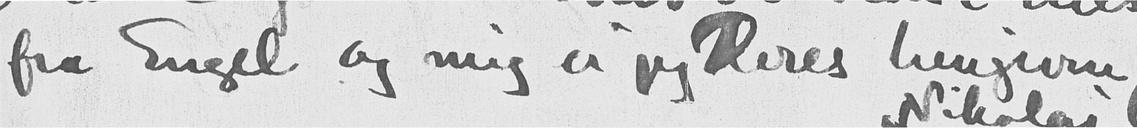fra Engel og mig er jeg Deres hengivne
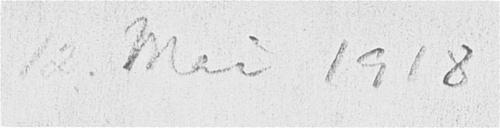12. mai 1918
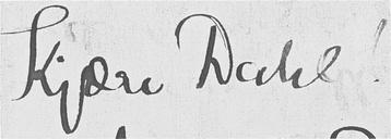Kjære Dahl!
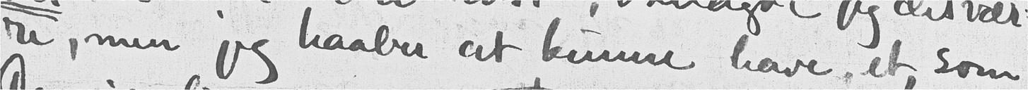re, men jeg haaber at kunne have, et, som
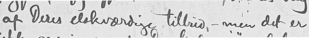af Deres elskværdige tilbud, - men det er
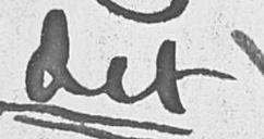det
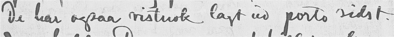De har ogsaa vistnok lagt ud porto sidst.
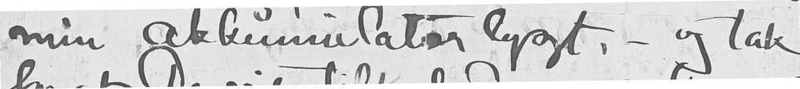min akkumulator lygt, - og tak
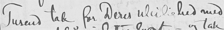Tusend tak for Deres uleilighed med
